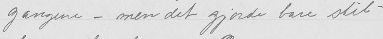gangene - men det gjorde bare stil-
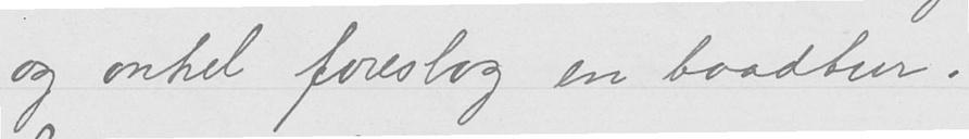og onkel foreslog en baadtur.
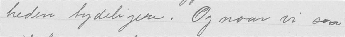heden tydeligere. Og naar vi saa
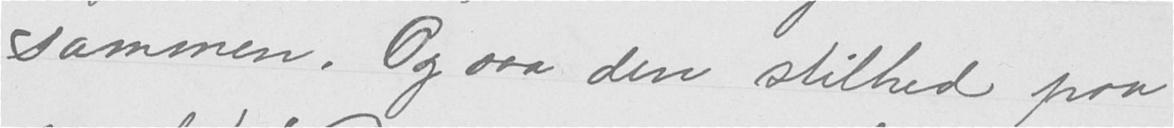sammen. Og saa den stilhed paa
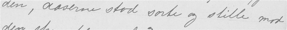den, aaserne stod sorte og stille mod
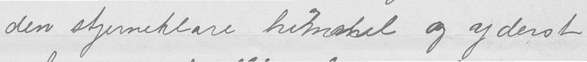den stjerneklare himmel og yderst
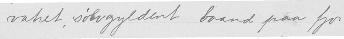vatret, sølvgyldent baand paa fjor-
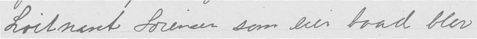Løitnant Sørensen som eier baad blev
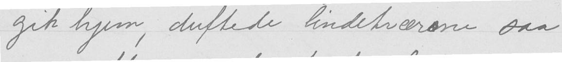gik hjem, duftede lindetrærne saa
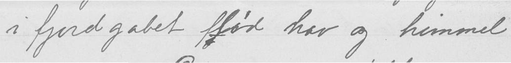i fjordgabet flød hav og himmel
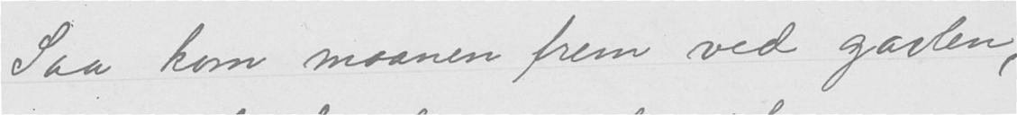Saa kom maanen frem ved gavlen,
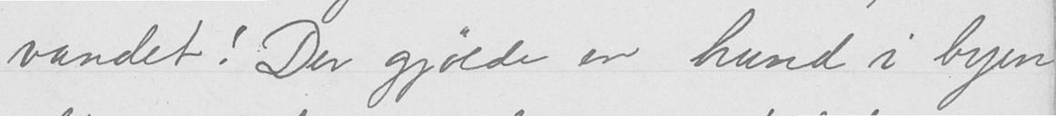vandet! Der gjøede en hund i byen
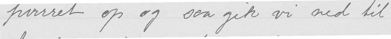purret op og saa gik vi ned til
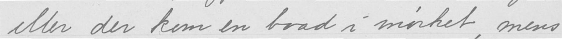eller der kom en baad i mørket, mens
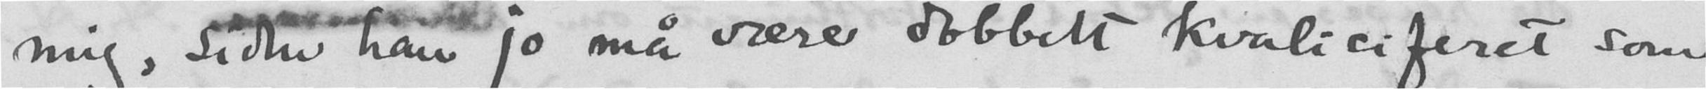mig, siden han jo må være dobbelt kvaliciferet som
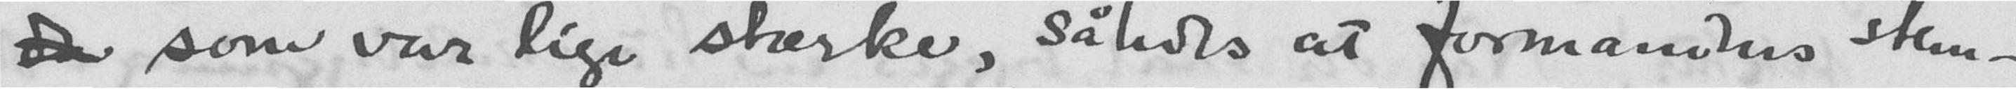da som var lige stærke, således at formandens stem-
